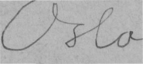Oslo
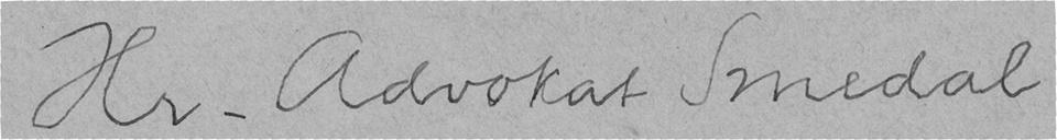Hr. Advokat Smedal
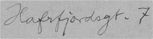Hafrfjordsgt. 7
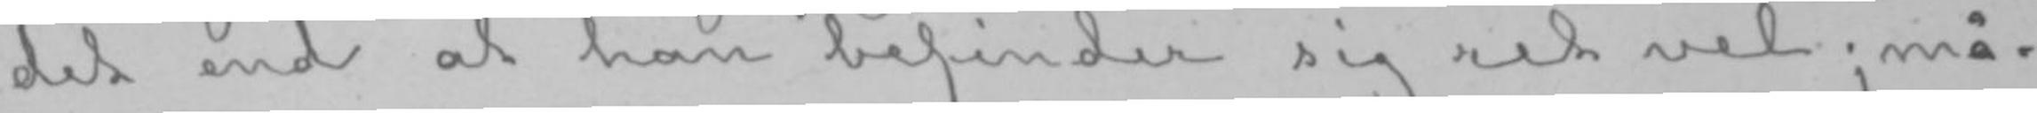det end at han befinder sig ret vel; må-
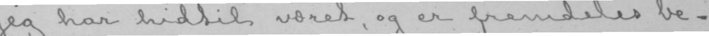Jeg har hidtil været, og er fremdeles be-
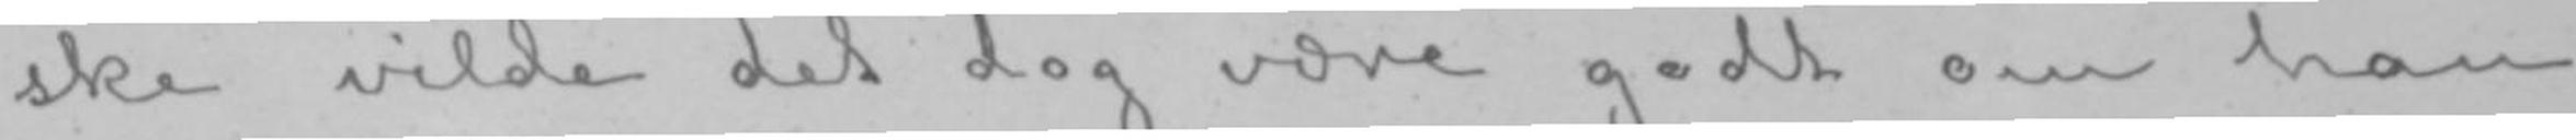ske vilde det dog være godt om han
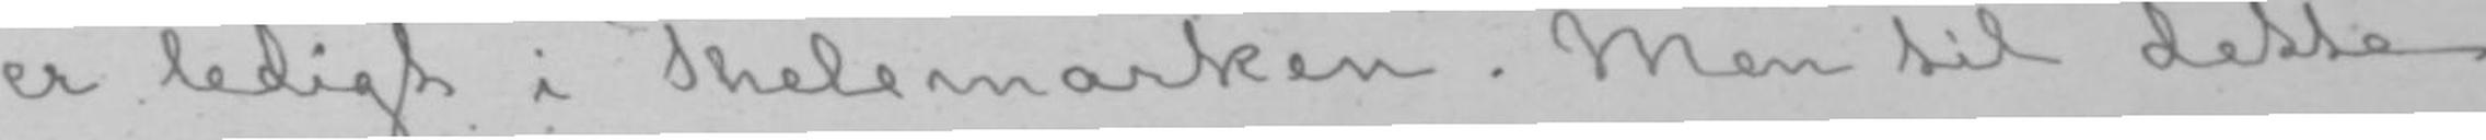er ledigt i Thelemarken. Men til dette
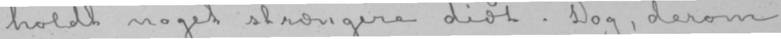holdt noget strængere diæt. Dog, derom
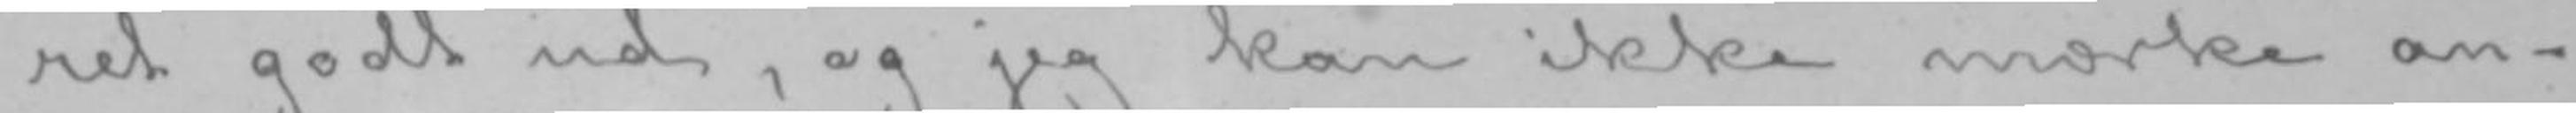ret godt ud, og jeg kan ikke mærke an-
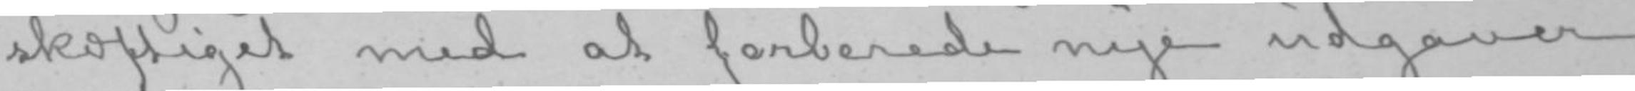skæftiget med at forberede nye udgaver
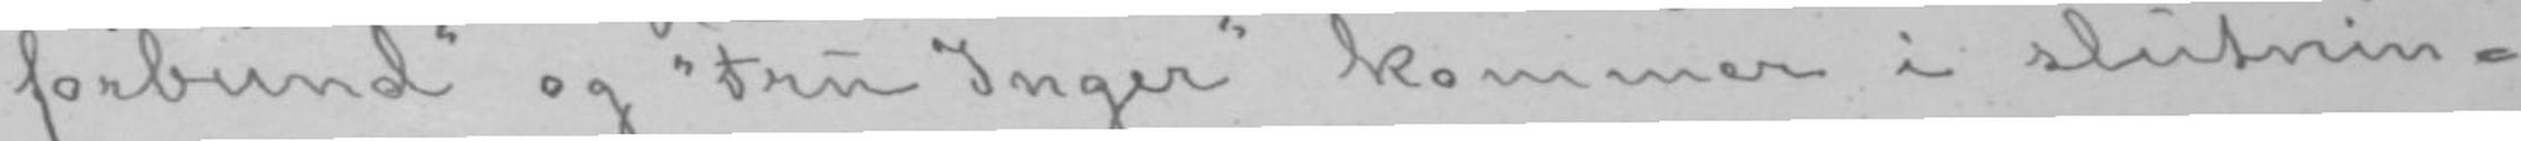forbund» og «Fru Inger» kommer i slutnin-
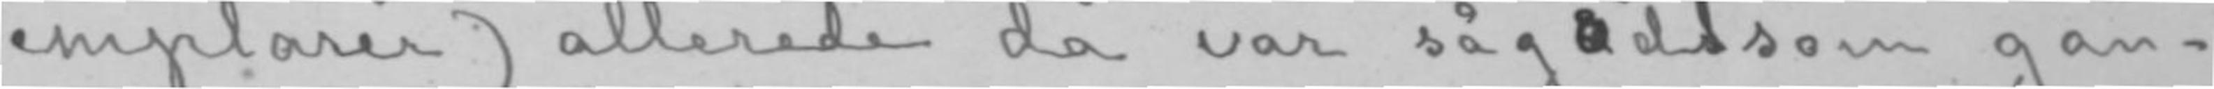emplarer) allerede da var sågodtsom gan-
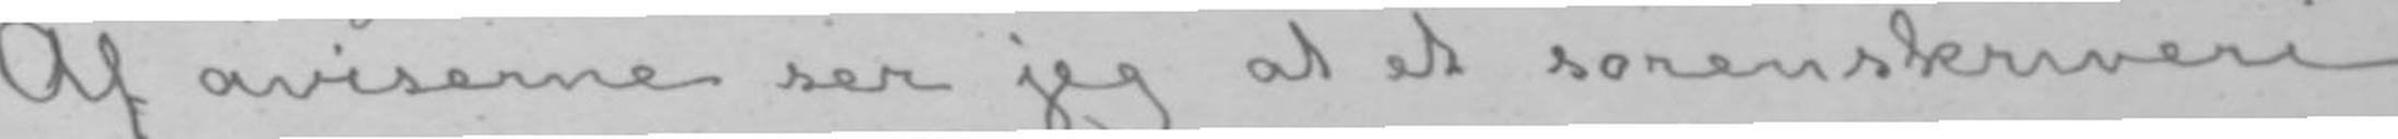Af aviserne ser jeg at et sorenskriveri
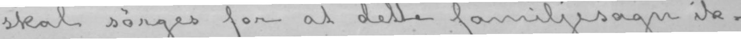skal sørges for at dette familjesagn ik-
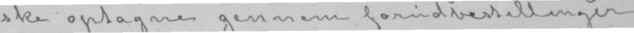ske optagne gennem forudbestillinger
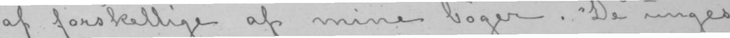af forskellige af mine bøger. «De unges
